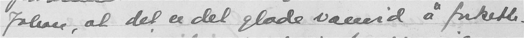Johan, at det er det glade vanvid å fortsette.
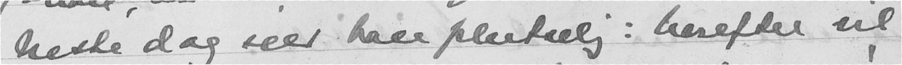Neste dag sier han plutselig: herefter vil
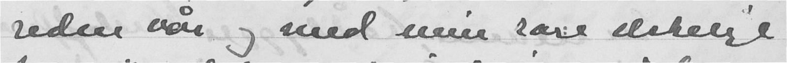reden vår og med min rare elskelige
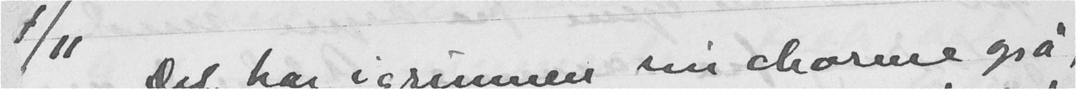1/11 Det har igrunnen sin charme også
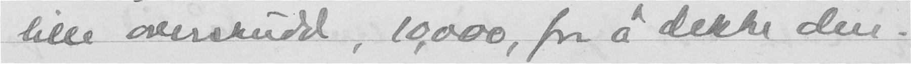lille overskudd, 10,000, for å dekke den.
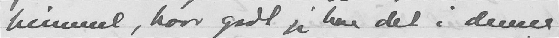himmel, hvor godt jeg har det i denne
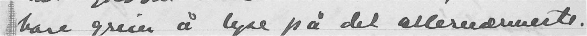bare greier å lyse på det allernærmeste.
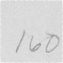160
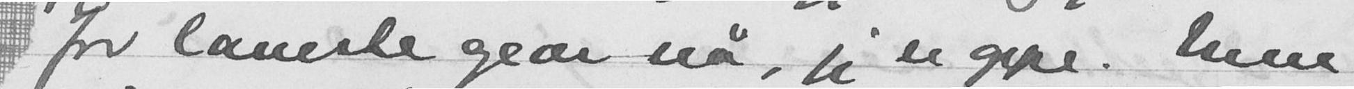for laveste gear nå, jeg er oppe. Men
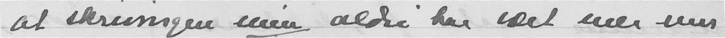at skrivingen min aldri har vært mer enn
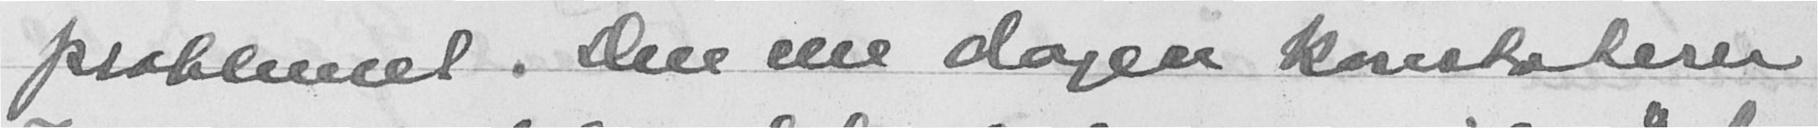problemet. Den ene dagen konstaterer
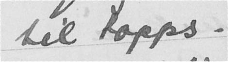til topps.
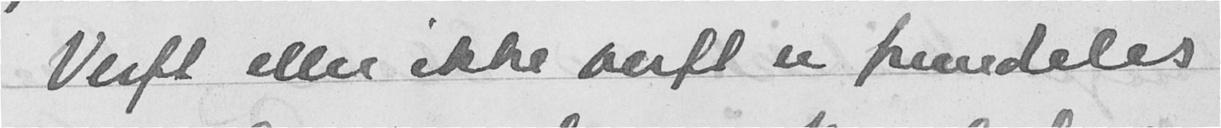Verft eller ikke verft er fremdeles
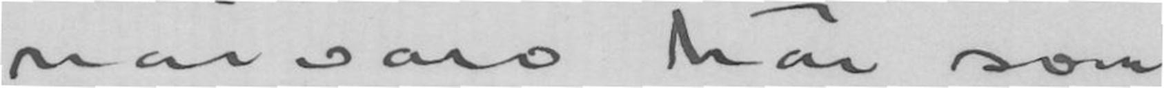närvaro här som
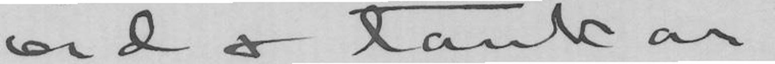ord & tankar
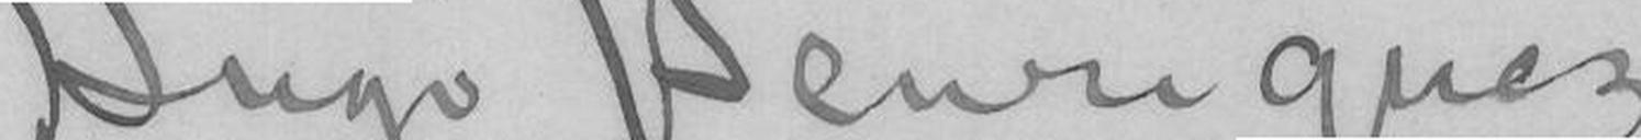Hugo Henriques
